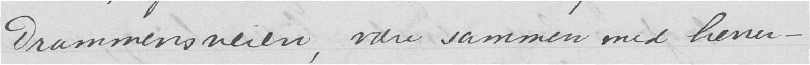Drammensveien, være sammen med herrer -
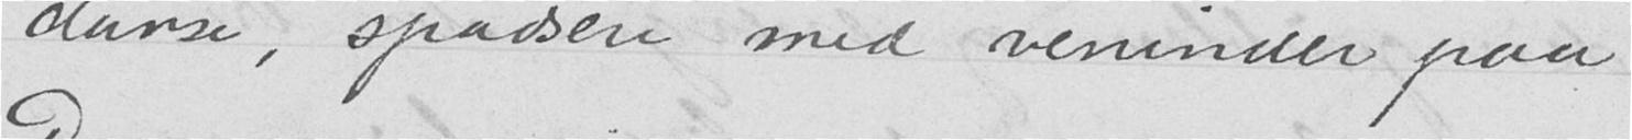danse, spadsere med veninder paa
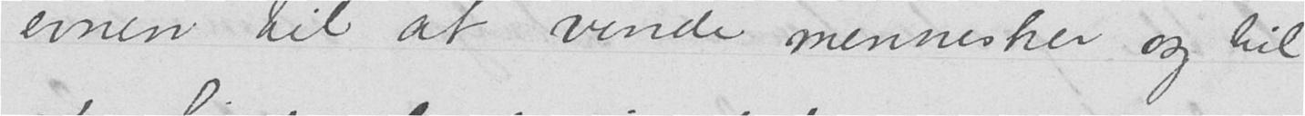evnen til at vinde mennesker og til
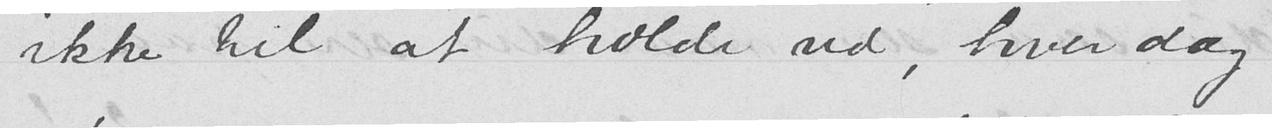ikke til at holde ud, hver dag
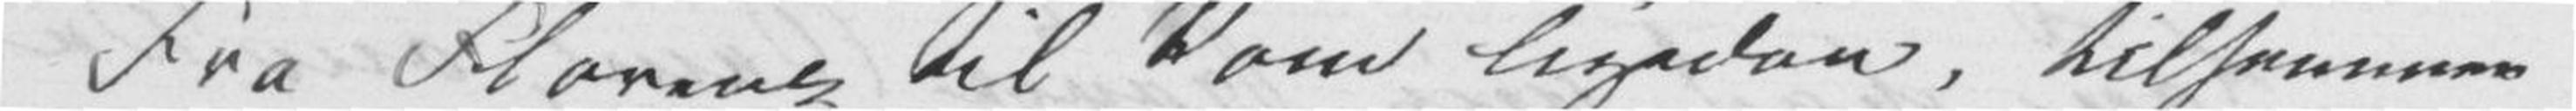Fra Florenz til Rom ligedan, tilsammen
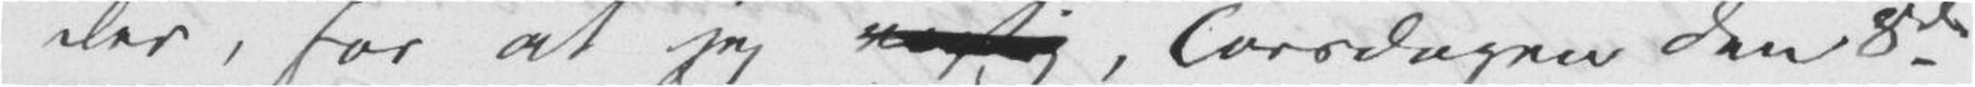der, for at jeg rigtig, Torsdagen den 8de
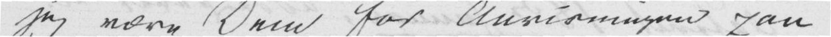jeg være Dem for Anvisningen paa
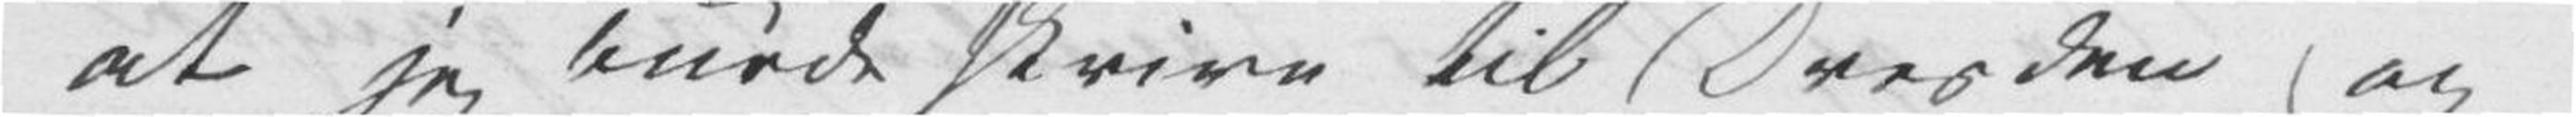at jeg burde skrive til Dresden og
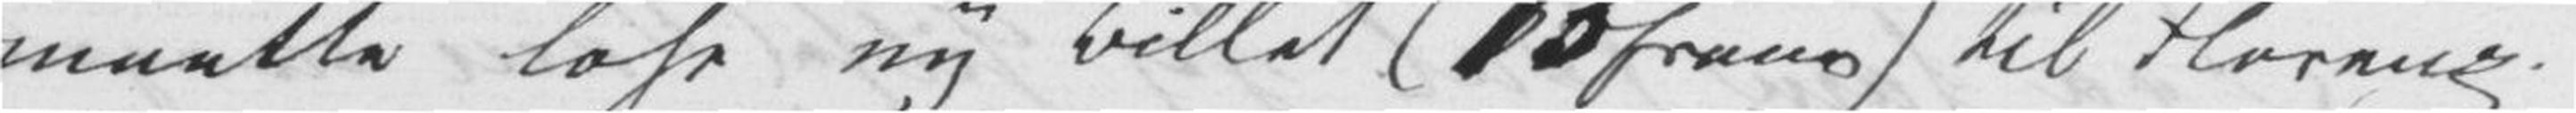maatte løse ny Billett (13 francs) til Florenz.
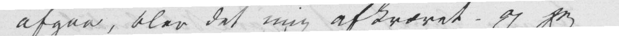afgaa, blev det mig afkrævet – og jeg
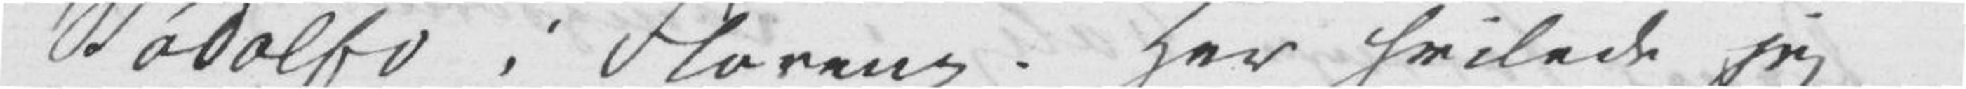Rodolfo i Florenz. Her hvilede jeg
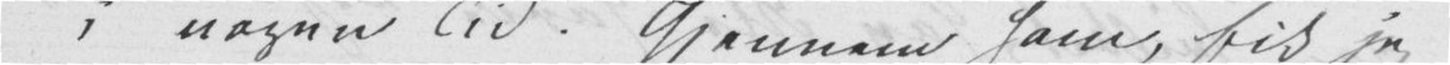i nogen Tid. Gjennem ham, fik jeg
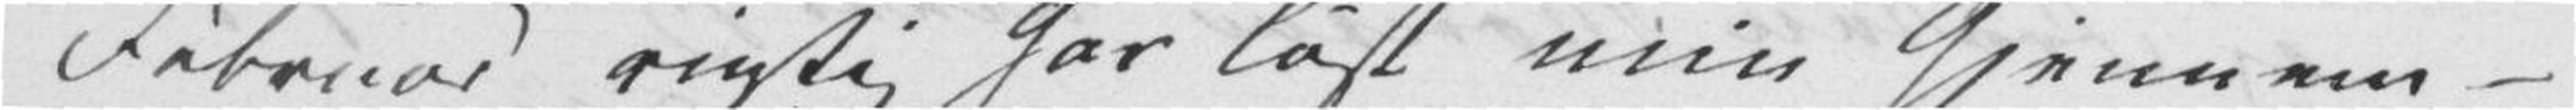Februar rigtig har løst min Gjennem¬
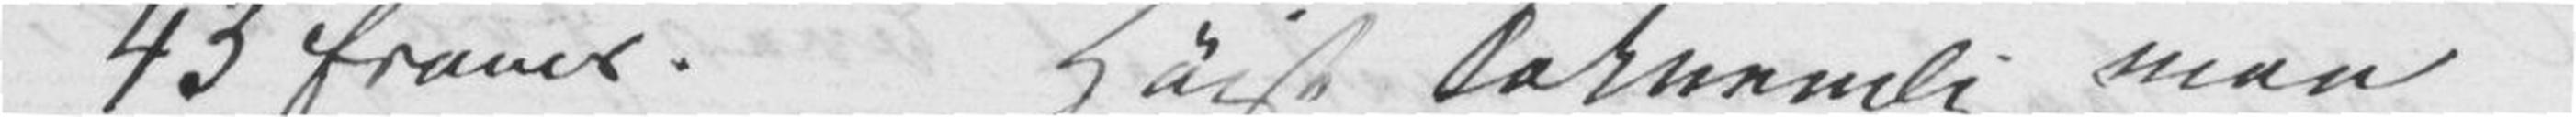43 francs. Høist taknemlig maa
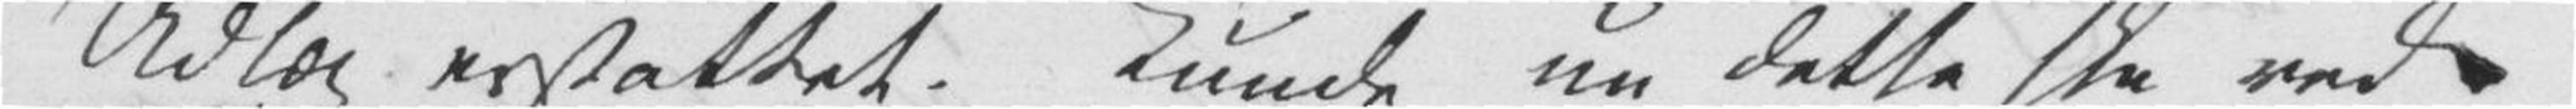Udlæg erstattet. Kunde nu dette ske ved
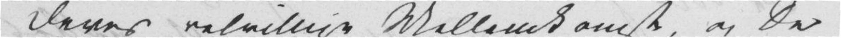Deres velvillige Mellemkomst, og De
